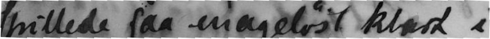spillede saa mageløst klart i
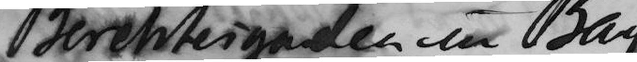Berehtesgaden in Bay-
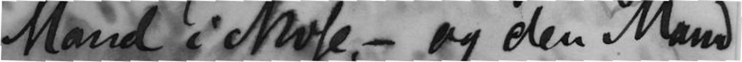Mand i Mose,- og den Mand
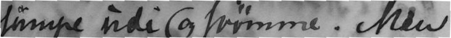jumpe ind i og svømme. Men
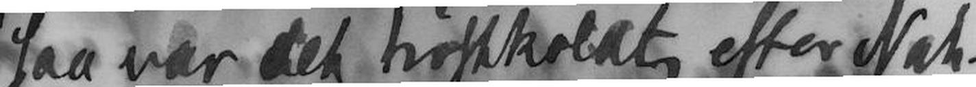saa var det høstkaldt efter Nat-
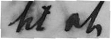til at
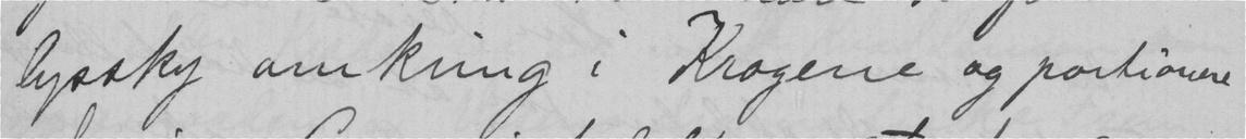lyssky omkring i Krogene og portionere
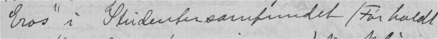Eros" i Studentersamfundet (For holdt
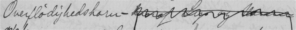Overflødighedshorn -
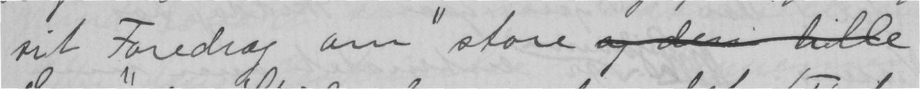sit Foredrag om "store
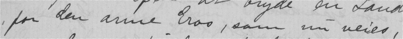for den arme Eros, som nu veies,
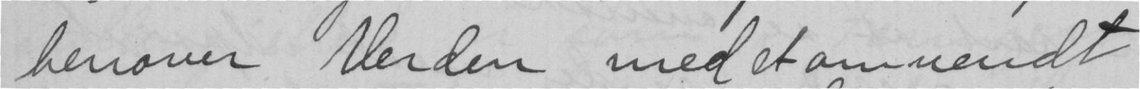henover verden med et omvendt
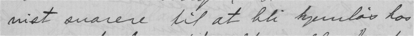vist snarere til at bli hjemløs hos
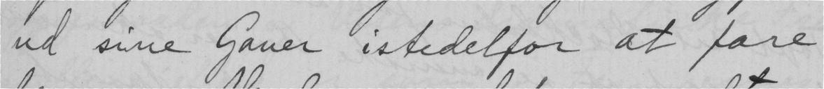ud sine Gaver istedelfor at fare
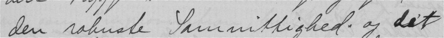den robuste Samvittighed og det
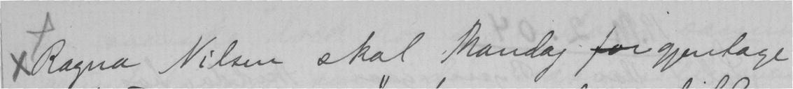x Ragna Nilsen skal mandag  gjentage
